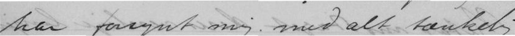har forsynt mig med alt tænkelig
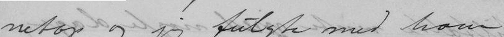netop og jeg fulgte med ham
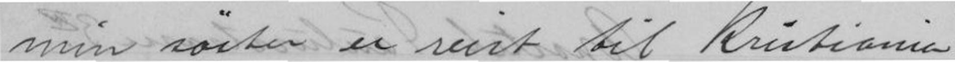min søster er reist til Kristiania,
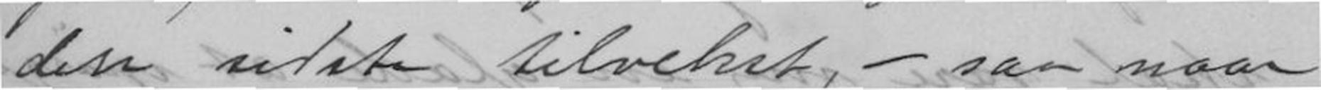den sidste tilvekst, - saa naar
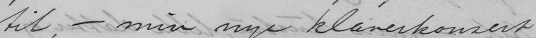til, - min nye klaverkonsert
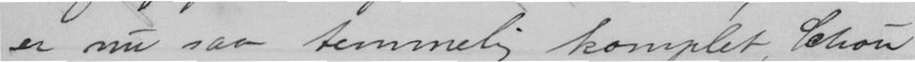er nu saa temmelig komplet, Schou
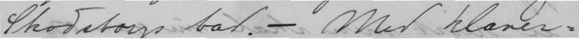Skodstorps bad. - Med klaver-
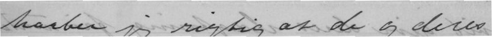haaber jeg rigtig at de og deres
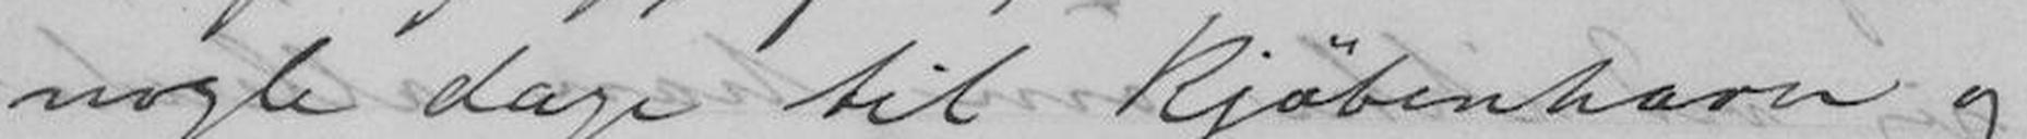nogle dage til Kjøbenhavn og
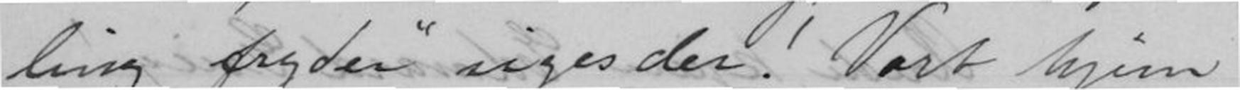ling fryder" siges det! Vort hjem
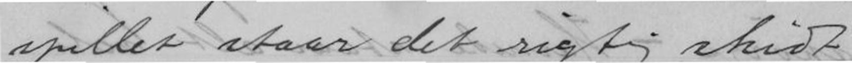spillet staar det rigtig skidt
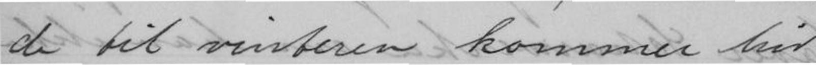de til vinteren kommer hid
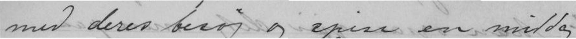med deres besøg og spise en middag
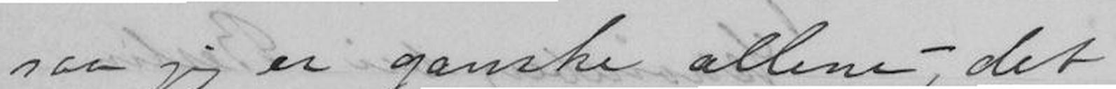saa jeg er ganske allene,- det
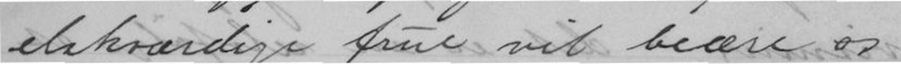elskværdige frue vil beære os
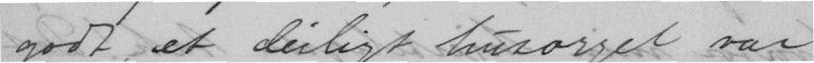godt, et deilig husorgel var
